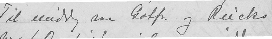Til middag var Gotfr. og Riecks
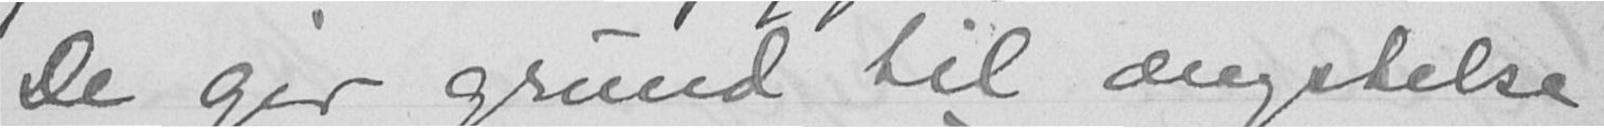De gir grund til ængstelse
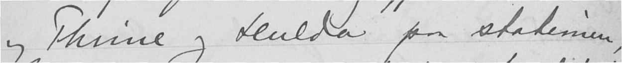og Thrine og Hulda paa stationen,
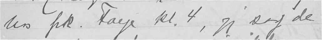hos frk. Faye kl. 4, jeg sagde
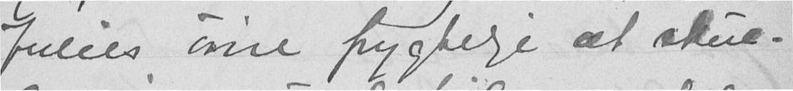Julies øine frygtelige at skue.
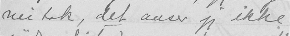nei tak, det anser jeg ikke
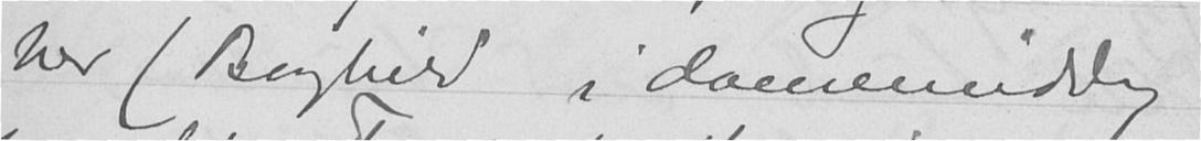her (Borghild i damemiddag
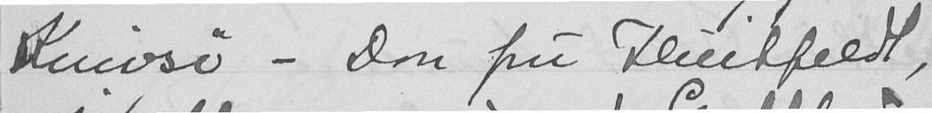Knivsø - Don fru Huitfeldt,
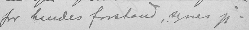for hendes forstand, synes jeg.
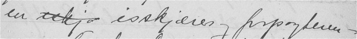en iskjo isskjærer og forpagteren -
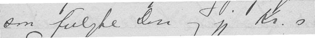som fulgte Don og jeg og Kr.s
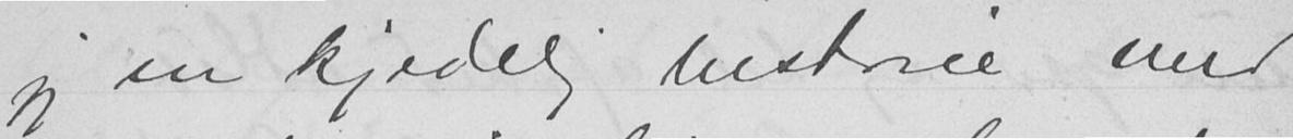jeg en kjædelig historie med
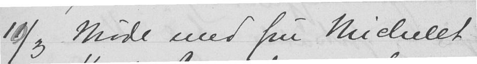10/3 Møde med fru Michelet
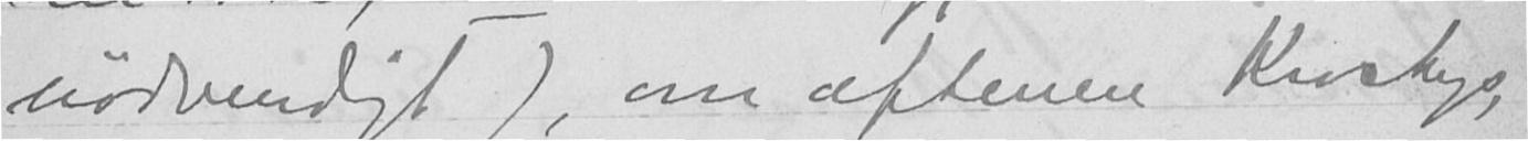nødvendigt), om aftenen Krosbys,
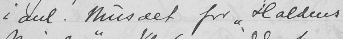i anl. Musæet for "Haldens
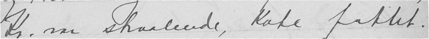Kr. var straalende, Kate fattet.
